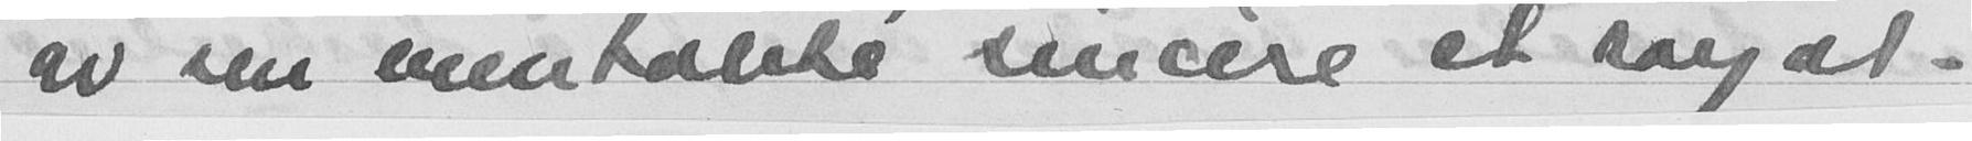av sin mentalité sincére et sayat.
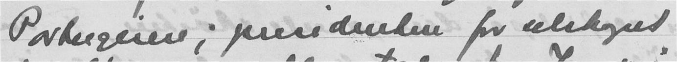Portugisere, presidenten for selskapet
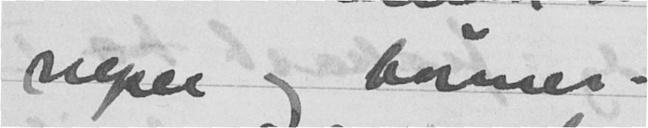neper og humser.
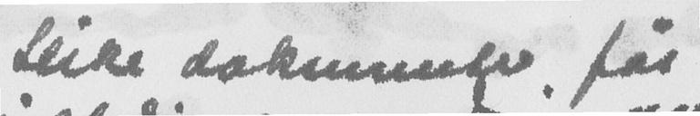Slike dokumenter får
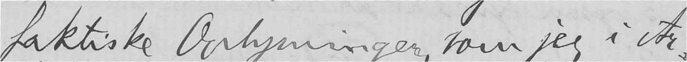faktiske Oplysninger, som jeg i Ar-
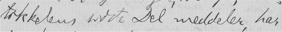tikkelens sidste Del meddeler, har
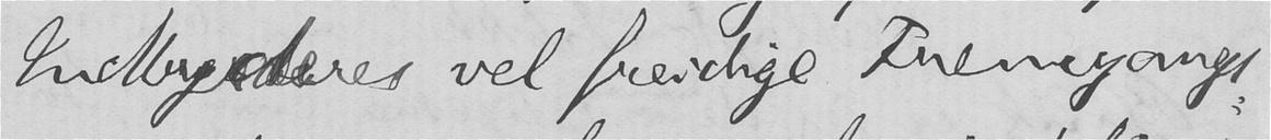Indbyderes vel freidige Fremgangs-
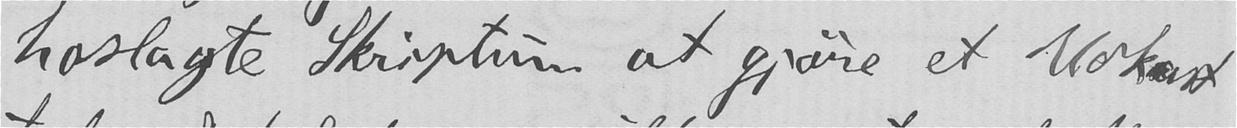hoslagte Skriptum at gjøre et Udkast
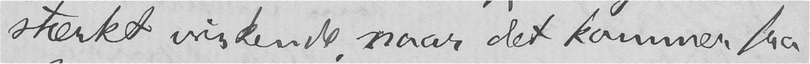stærkt virkende, naar det kommer fra
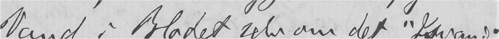Vand i Blodet, selv om det "Isvand",
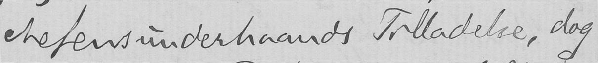chefens underhaands Tilladelse, dog
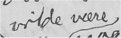vilde være
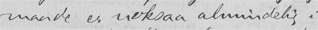maade er noksaa almindelig i
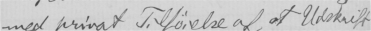med privat Tilføielse af, at Udskrift
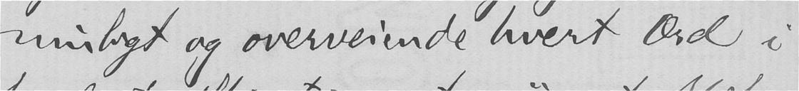muligt og overveiende hvert Ord i
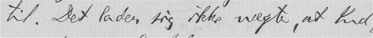til. Det lader sig ikke nægte, at Ind-
